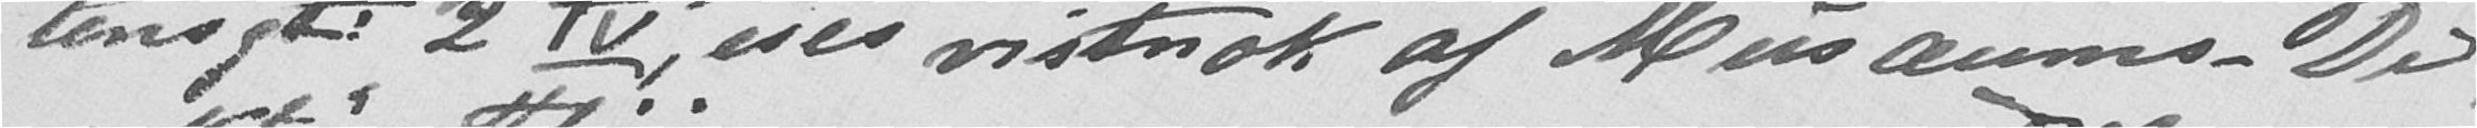tensgt. 2 IV, eies vistnok af Musæums-Di-
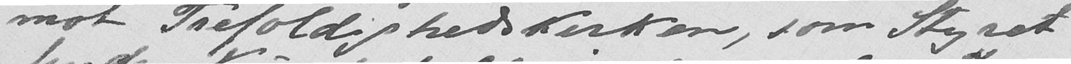mot Trefoldighedskirken, som Styret
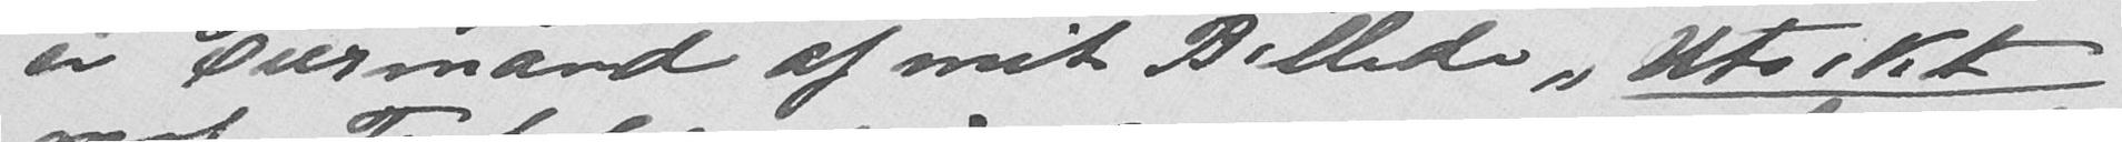er Eiermand af mit Billede "Utsikt
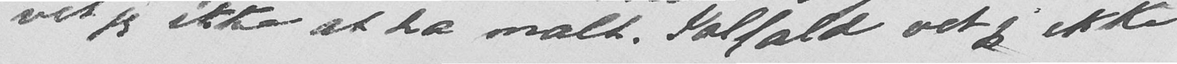vet jeg ikke at ha malt. Ialfald vet jeg ikke
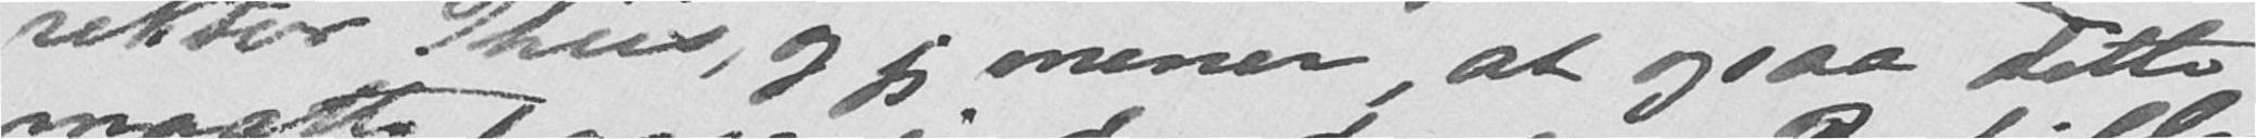rektør Thiis, og jeg mener, at ogsaa dette
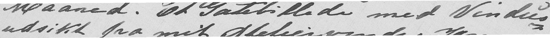Maaned. Et Gatebillede med Vindus-
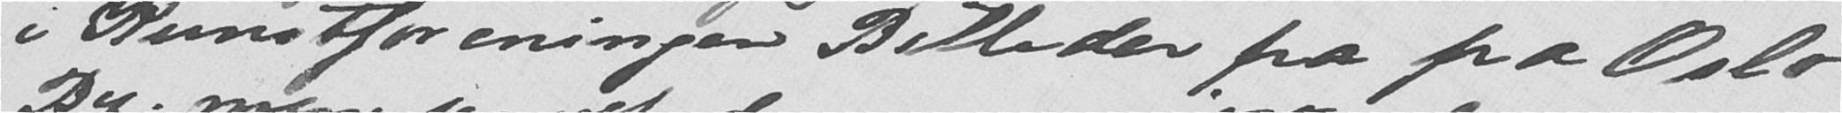i Kunstforeningen Billeder fra fra Oslo
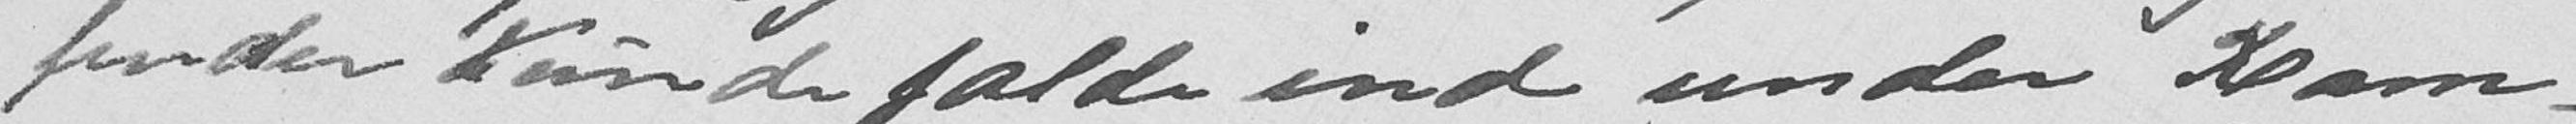finder kunde falde ind under Ram-
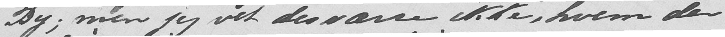By; men jeg vet desværre ikke, hvem der
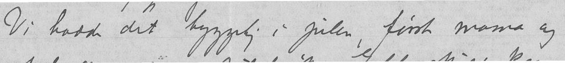Vi hadde det hyggelig i julen, først mama og
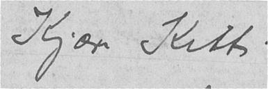Kjære Kitti,
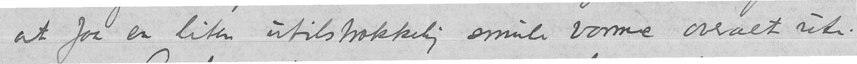at faa en liten utilstrækkelig smule varme overalt ute.
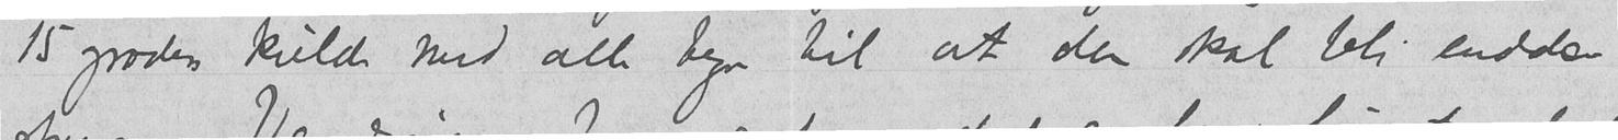15 graders kulde med alle tegn til at den skal bli endda
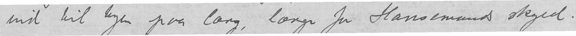ind til byen paa længe, længe for Hansemands skyld.
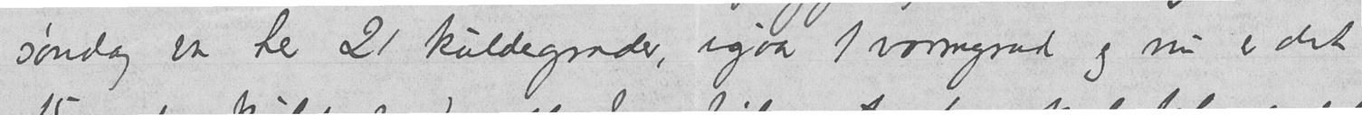søndag var her 21 kuldegrader, igaar 1 varmgrad og nu er det
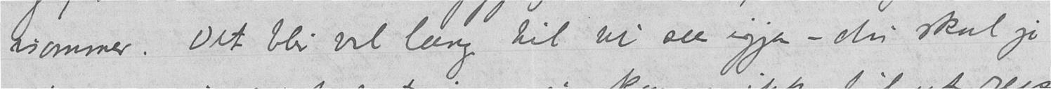isommer. Det blir vel længe til vi ses igjen – du skal jo
